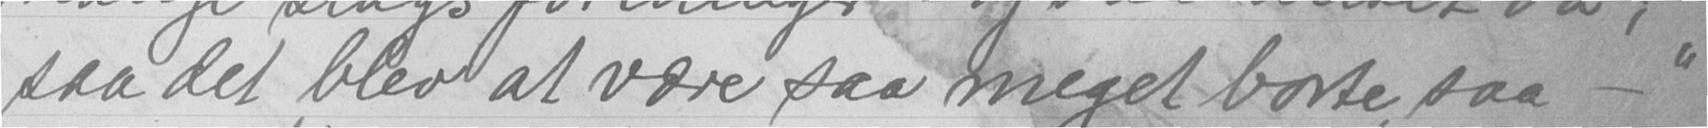saa det blev at være saa meget borte, saa - "
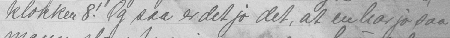klokken 8! Og saa er det jo det, at en har jo saa
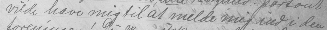vilde have mig til at melde mig ind i den
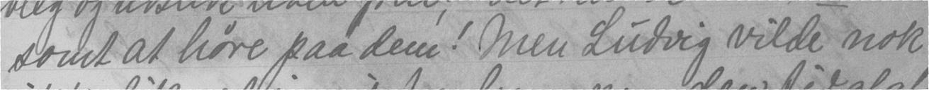somt at høre paa dem! Men Ludvig vilde nok
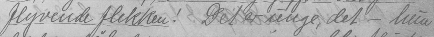flyvende flekken! Det er unge, det - hun
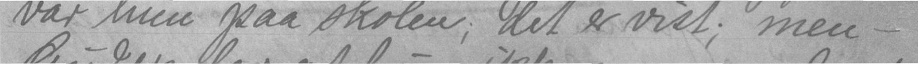var hun paa skolen; det er vist; men -
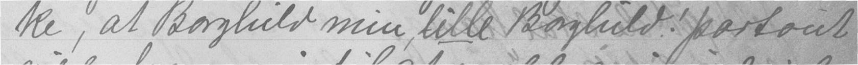ke, at Borghild min, lille Borghild! partont
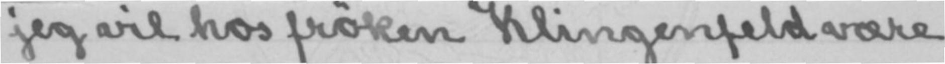jeg vil hos frøken Klingenfeld være
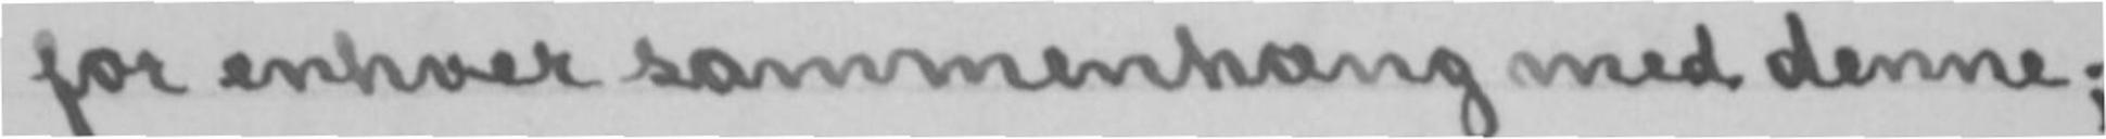for enhver sammenhæng med denne;
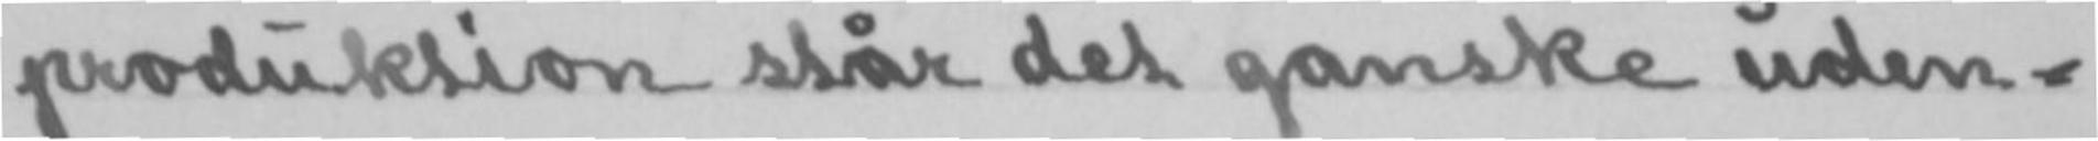produktion står det ganske uden-
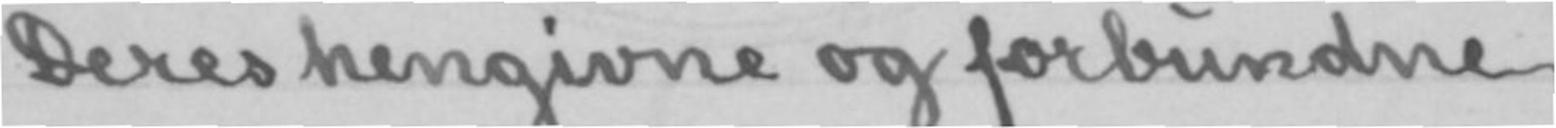Deres hengivne og forbundne
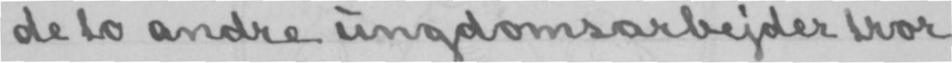de to andre ungdomsarbejder tror
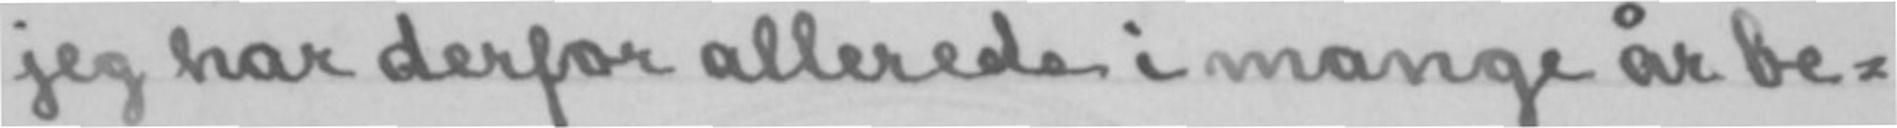jeg har derfor allerede i mange år be-
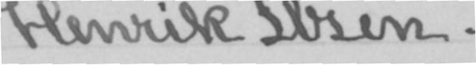Henrik Ibsen.
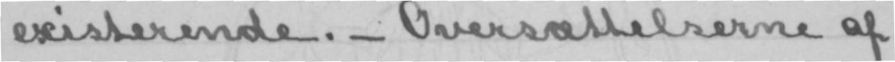existerende.- Oversættelserne af
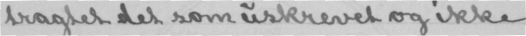tragtet det som uskrevet og ikke
